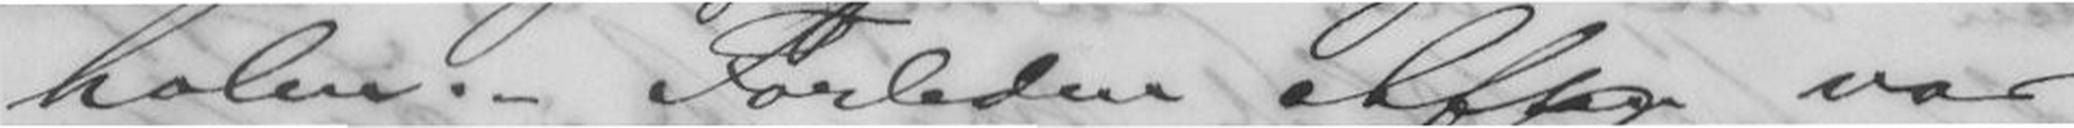holm. - Forleden Aften var
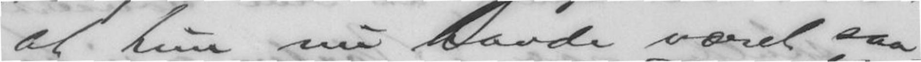at hun nu havde været saa-
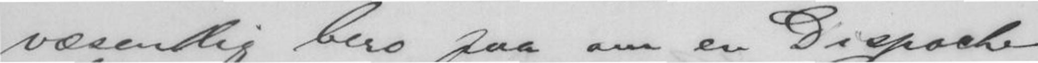væsentlig bero paa om en Dispache
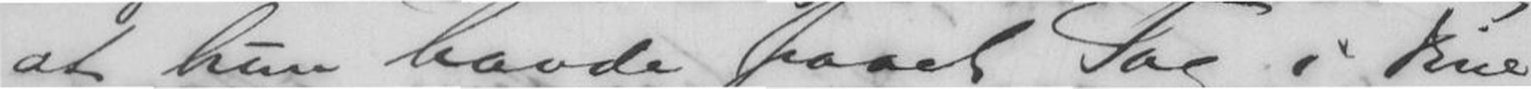at hun havde faaet Tag i Dine
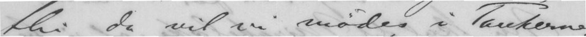thi da vil vi mødes i Tankerne.
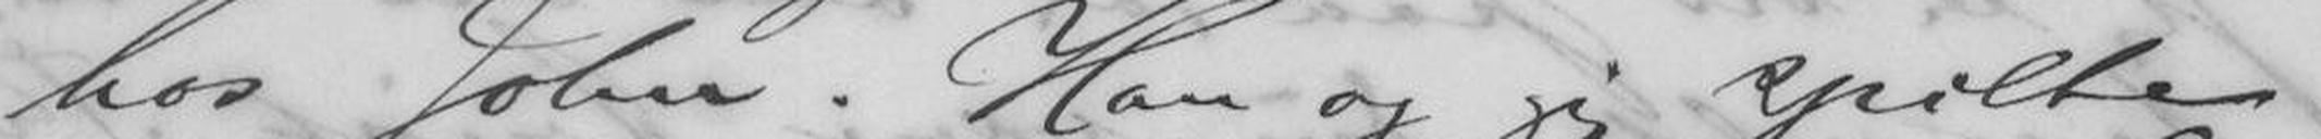hos John. Han og jeg spilte
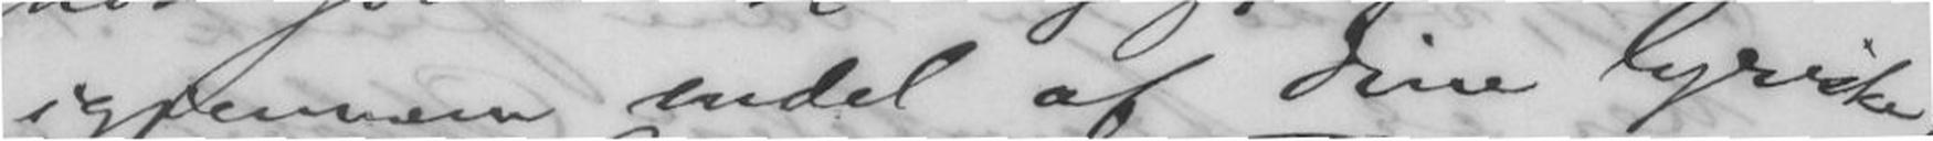igjennem endel af dine lyriske,
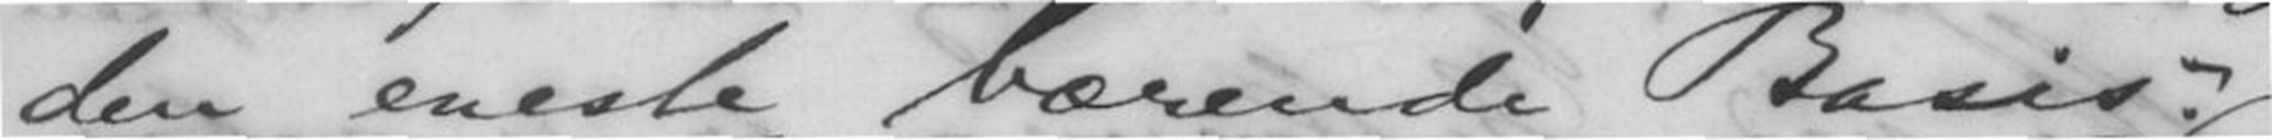den eneste bærende Basis.
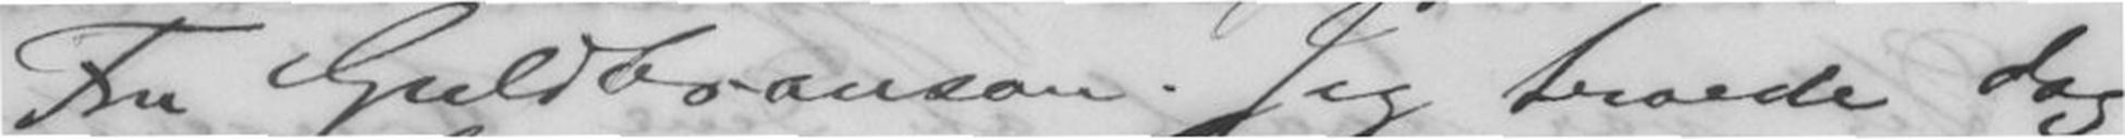Fru Guldbranson. Jeg troede dog,
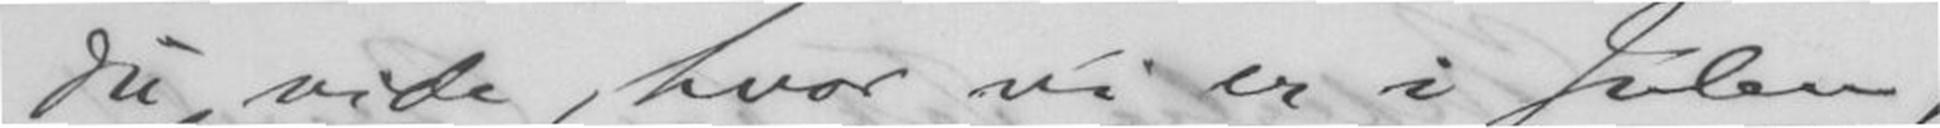du vide, hvor vi er i Julen,
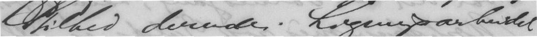Stilhed derude. Ligningsarbeidet
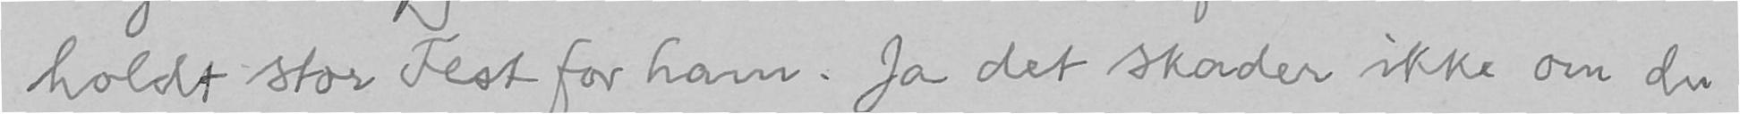holdt stor Fest for ham. Ja det skader ikke om du
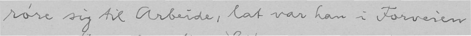røre sig til Arbeide, lat var han i Forveien
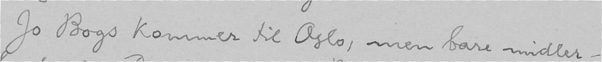Jo Bogs kommer til Oslo, men bare midler-
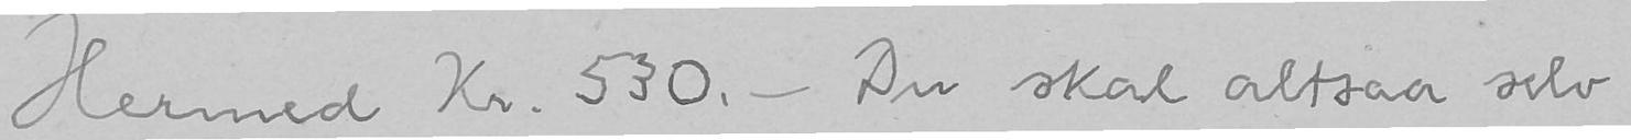Hermed Kr. 530. - Du skal altsaa selv
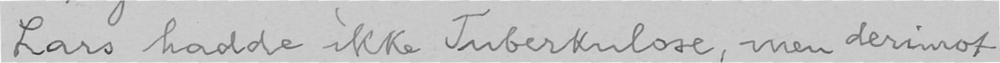Lars hadde ikke Tuberkulose, men derimot
